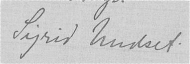Sigrid Undset.
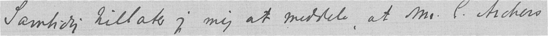Samtidig tillater jeg mig at meddele, at Mr. C. Archers
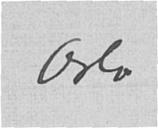Oslo
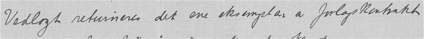Vedlagt returneres det ene eksemplar av forlagskontrakten
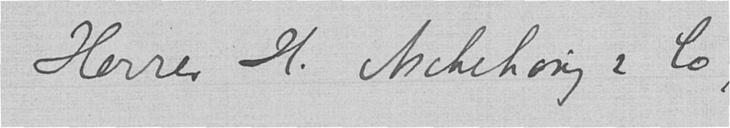Herrer H. Aschehoug & Co,
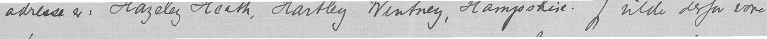adresse er: Hazeley Heath, Hartley Wintney, Hampshire. Jeg vilde derfor være
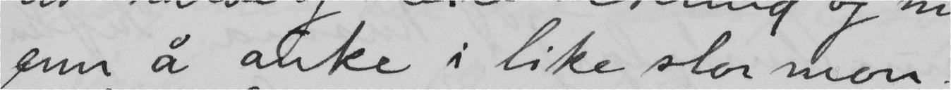enn å anke i like stor mon
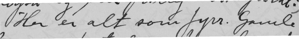Her er alt som fyrr. Gamle
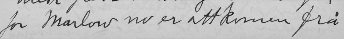for Marlow no er attkomen frå
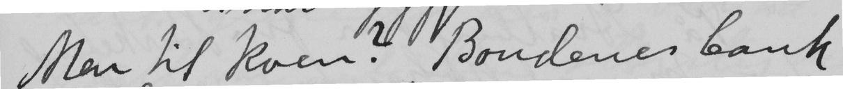Men til kven? Bondenes bank
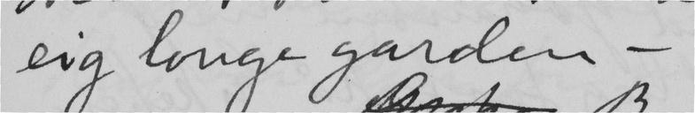eig longe garden –
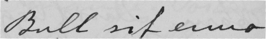Bull sit enno
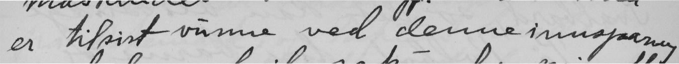er tilsist vunne ved denne innsprang
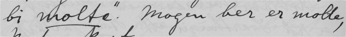bi molte". Mogen ber er molte,
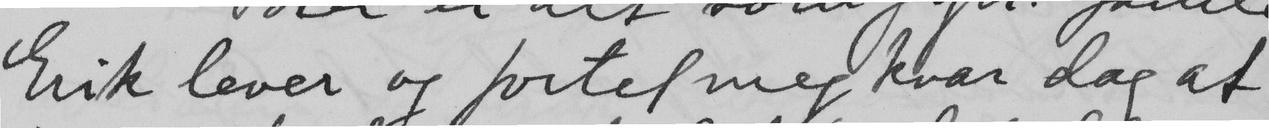Erik lever og fortel meg kvar dag at
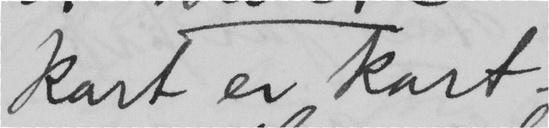kart er kart –
